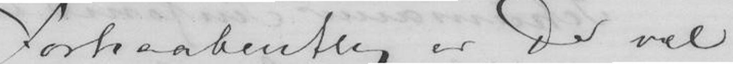Forhaabentlig er De vEl
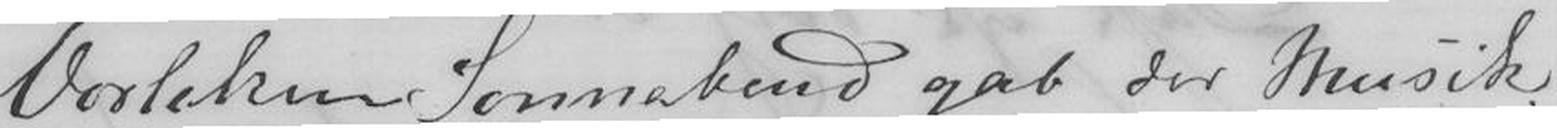Vorleben Sonnabend gab der Musik-
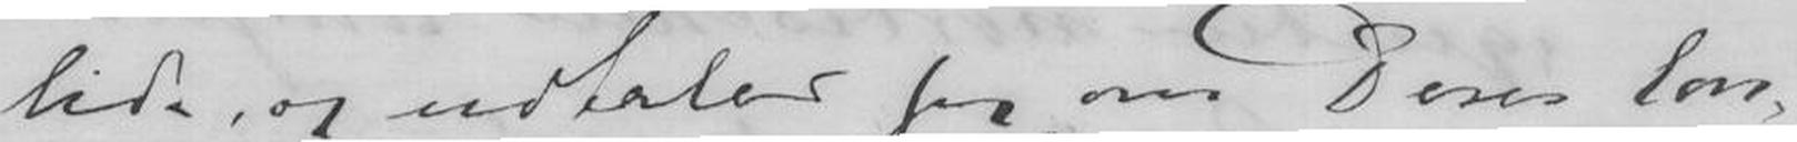lidt og udtaler sig om Deres Con-
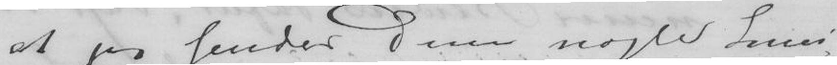at jeg sender Dem nogle Lini-
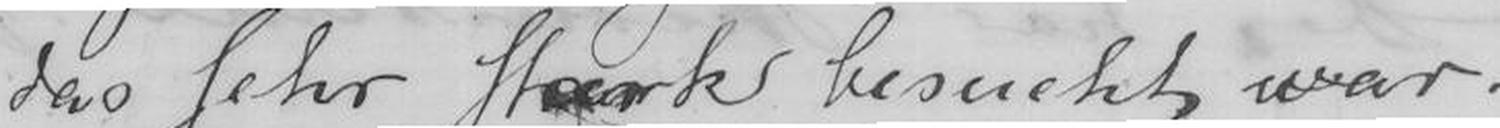das sehr stark besucht war.
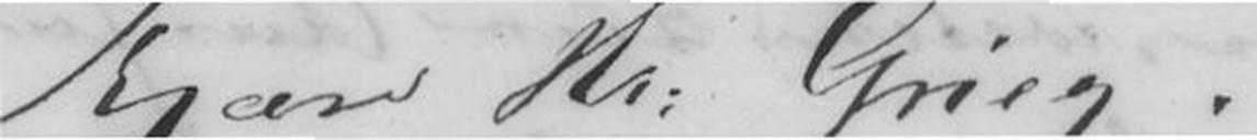Kjære Hr. Grieg!
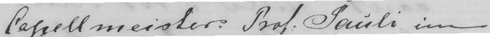Capellmeister Prof Pauli im
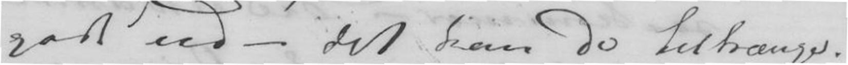godt ud - det kan De tiltrænge
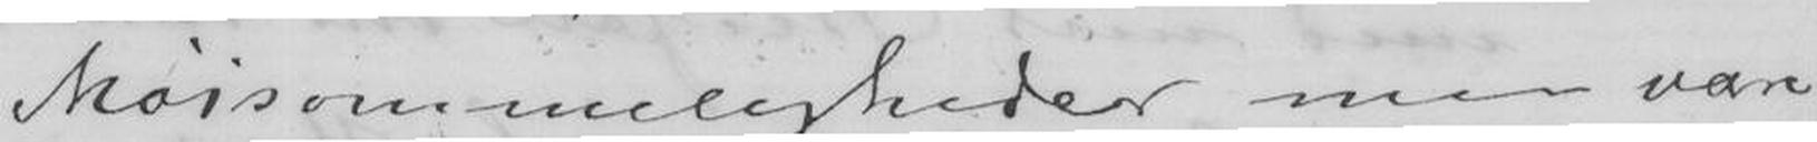Møisommeligheder maa være
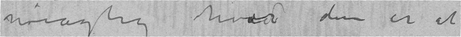nøyagtig hvor den er at
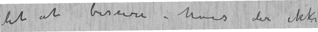let at beseire – Hvis der ikke
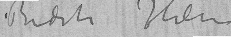Bedste Hilsen
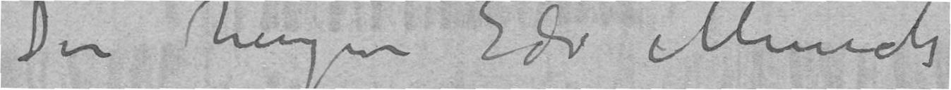Din hengivne Edv Munch
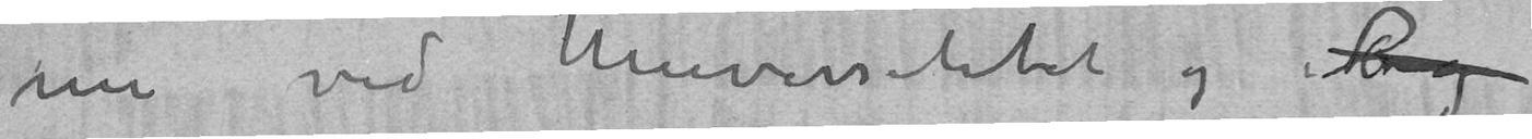nu ved Universitetet og
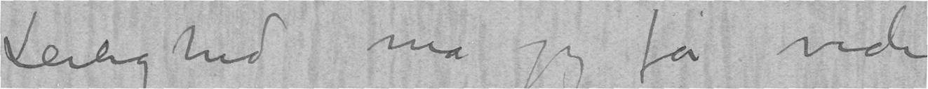Leilighed må jeg få vide
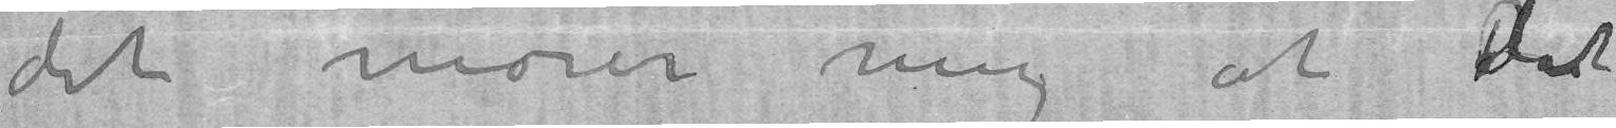det morer mig at det
